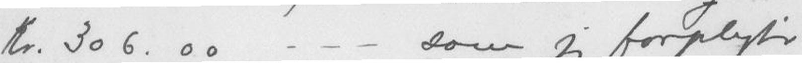Kr. 306.00 - - - som jeg forpligter
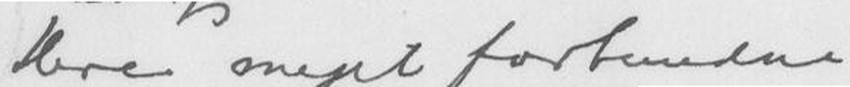Deres meget forbundne
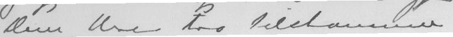Dem Deres tro tilskamme.
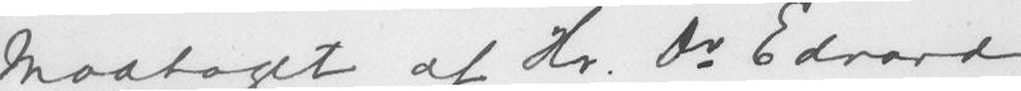Modtaget af Hr. Dr Edvard
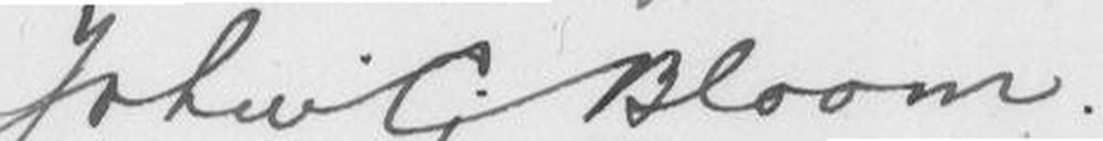John G. Bloom
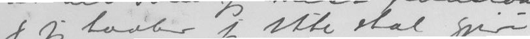og jeg haaber jeg ikke skal gjøre
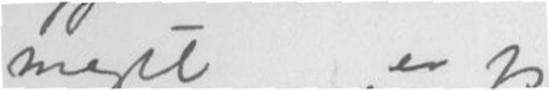megeter jeg
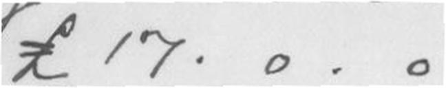£17.o.o
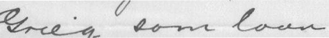Grieg som laan
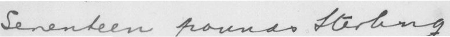Senenteen pounds Sterling
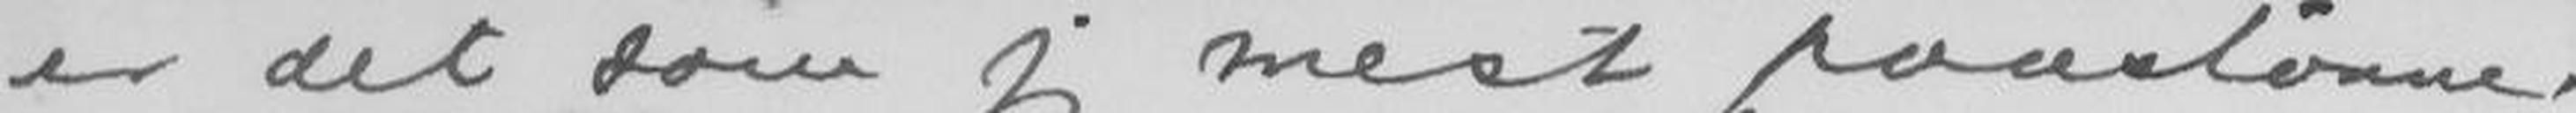er det jeg mest paaskønne
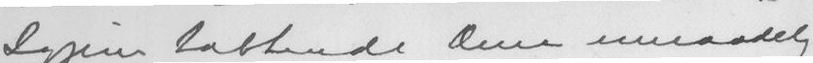Igjen takkende Dem umaadelig
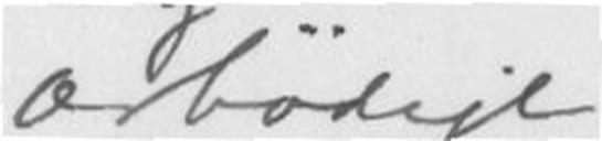ærbødige
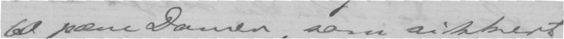60 pæne Damer, som sikkert
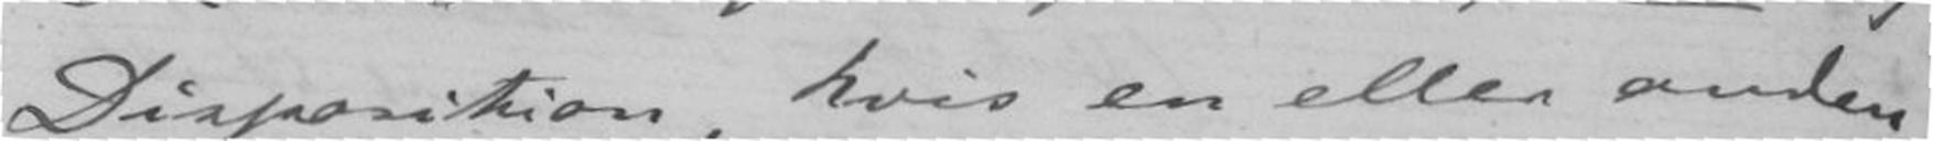Disposition, hvis en eller anden
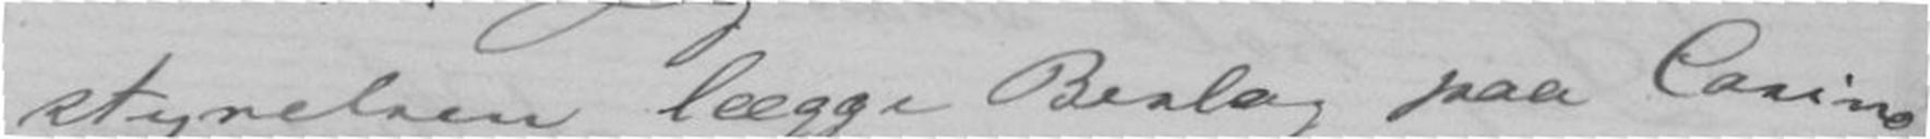styrelsen lægge beslag paa Casino
